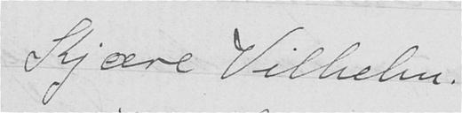Kjære Vilhelm!
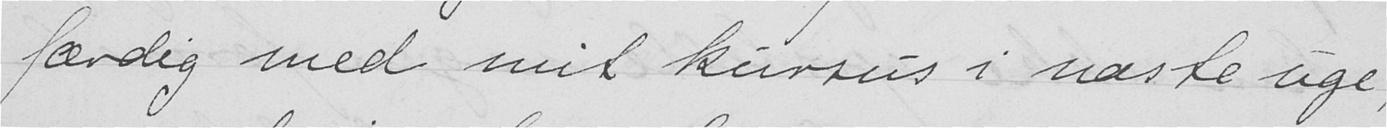færdig med mit kursus i næste uge,
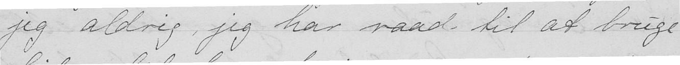jeg aldrig, jeg har raad til at bruge
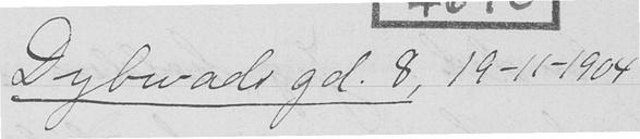Dybvads gd. 8, 19-11-1904
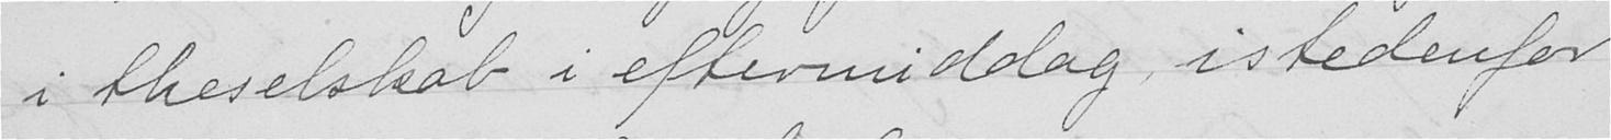i theselskab i eftermiddag, istedenfor
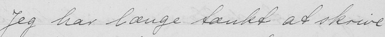Jeg har længe tænkt at skrive
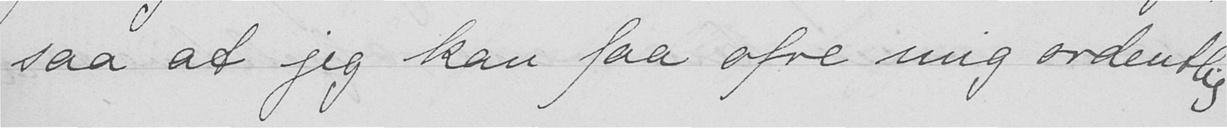saa at jeg kan faa ofre mig ordentlig
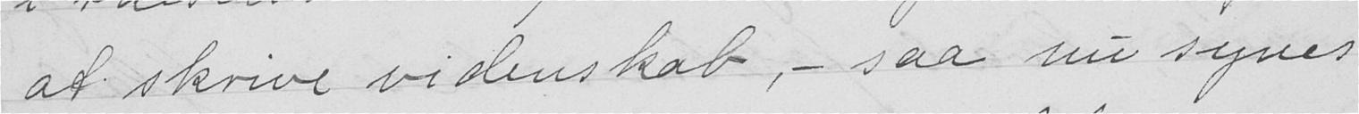at skrive videnskab, - saa nu synes
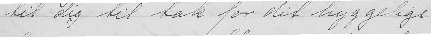til dig til tak for det hyggelige
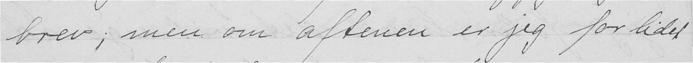brev; men om aftenen er jeg for lidet
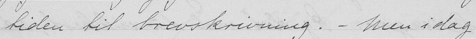tiden til brevskrivning. - Men i dag
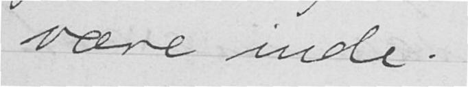være inde.
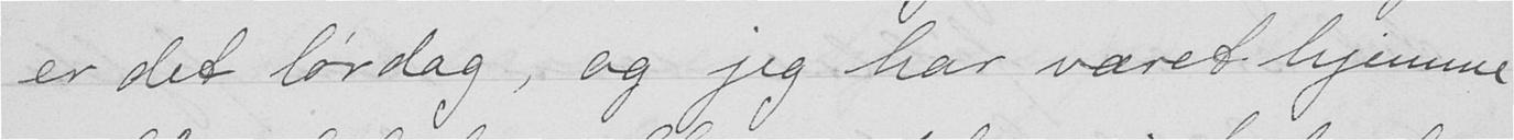er det lørdag, og jeg har været hjemme
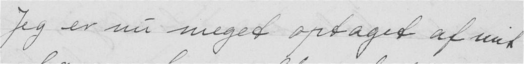Jeg er nu meget optaget af mit
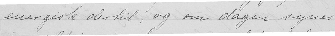energisk dertil, og om dagen synes
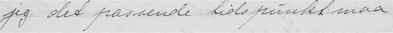jeg det passende tidspunkt maa
